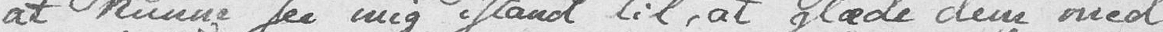at kunne see mig istand til, at glæde dem med
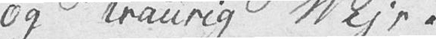og traurig Vejr.
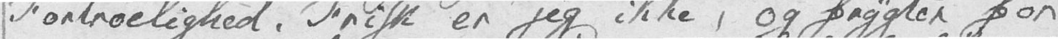Fortroelighed. Frisk er jeg ikke, og frygter for
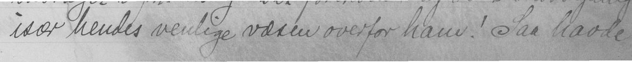især hendes venlige væsen overfor ham! Saa havde
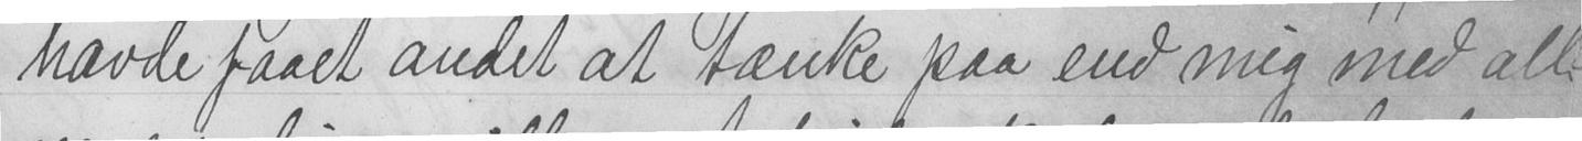havde faaet andet at tænke paa end mig med all
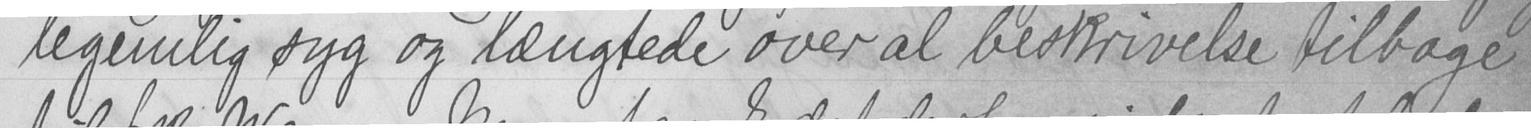legemlig syg og længtede over al beskrivelse tilbage
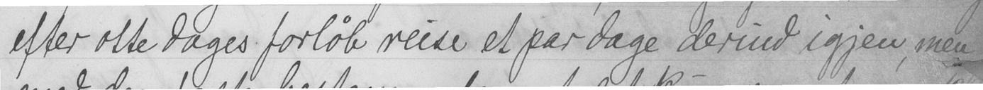efter otte dages forløb reise et par dage derind igjen, men
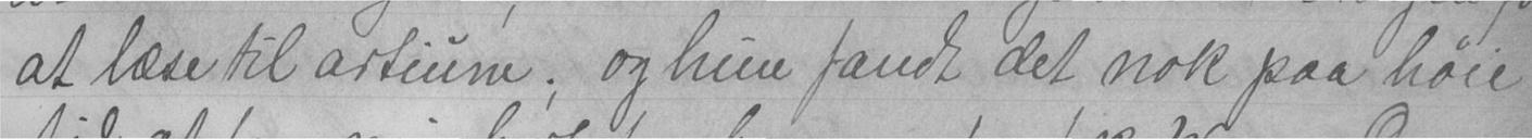at læse til artium; og hun fandt det nok paa høie
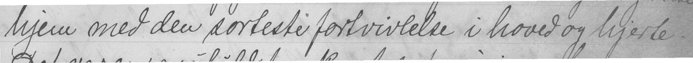hjem med den sorteste fortvivlelse i hoved og hjerte.
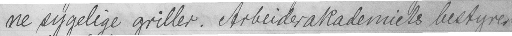ne sygelige griller. Arbeiderakademiets bestyrer
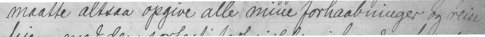maatte altsaa opgive alle mine forhaabninger og reise
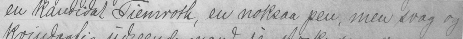en kandidat Tiemroth, en noksaa pen, men svag og
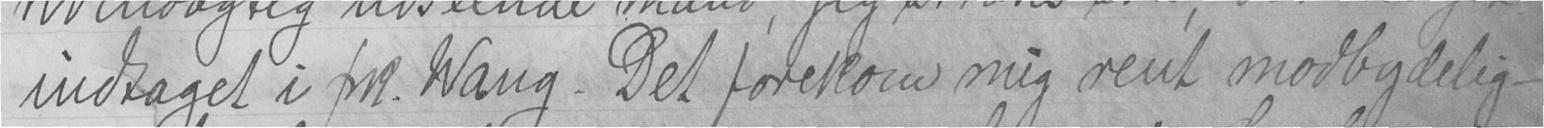indtaget i frk. Wang. Det forekom mig rent modbydelig -
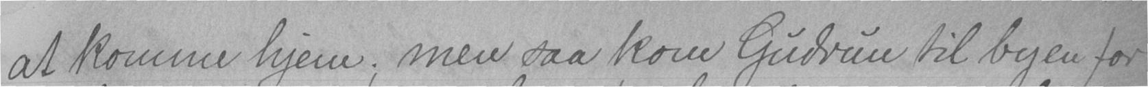at komme hjem; men saa kom Gudrun til byen for
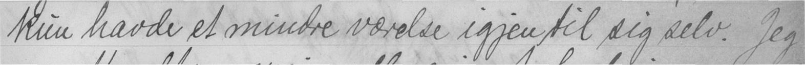kun havde et mindre værelse igjen til sig selv. Jeg
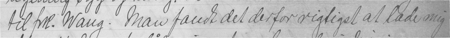til frk. Wang. Man fandt det derfor rigtigst at lade mig
